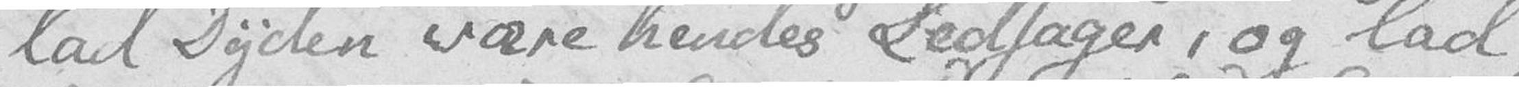lad Dyden være hendes Ledsager, og lad
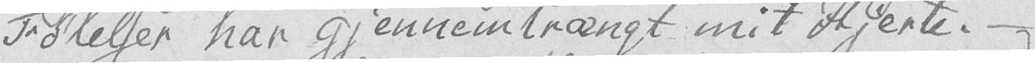Følelser har gjennemtrængt mit Hjerte. –
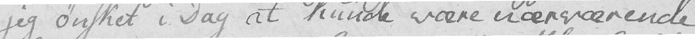jeg ønsket i Dag at Kunde være nærværende
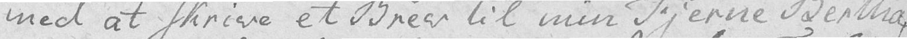med at skrive et Brev til min Fjerne Bertha,
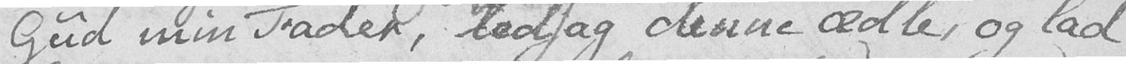Gud min Fader, ledsag denne ædle, og lad
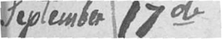September 17de
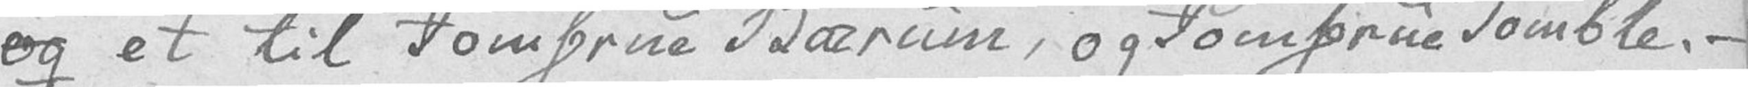og et til Jomfrue Bærum, og Jomfrue Tomble. –
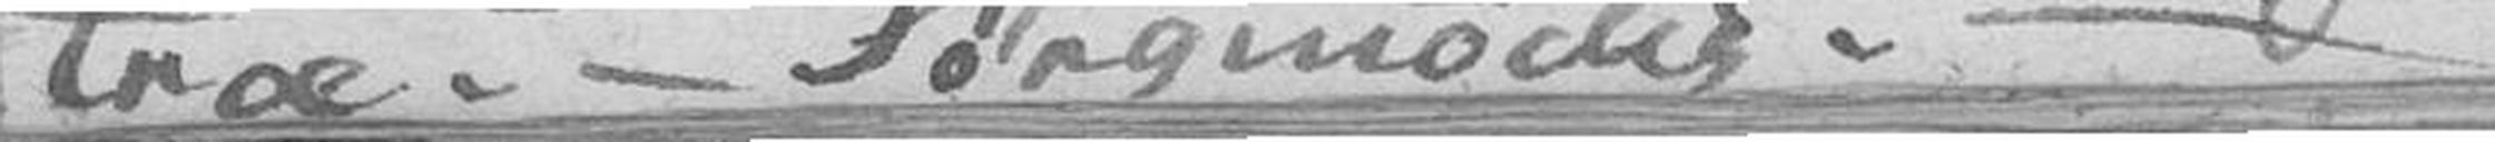troe. – Sørgmodig. –
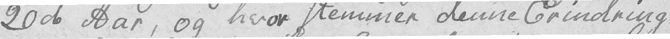20de Aar, og hvor stemmer denne Erindring
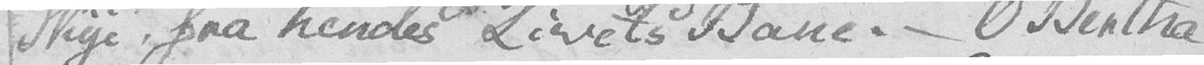Skye, fra hendes Livets Bane. – O Bertha
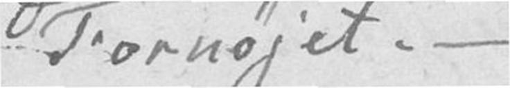Fornøjet. –
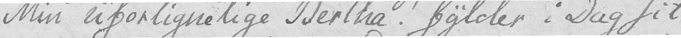Min Uforlignenlige Bertha! fylder i Dag sit
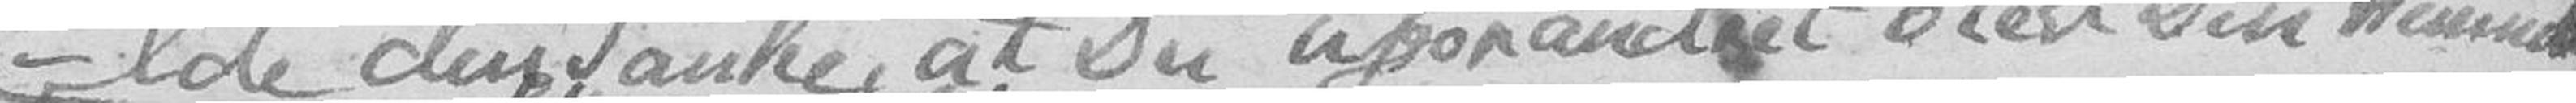-lde den Tanke, at Du uforandret blev Din Veninde
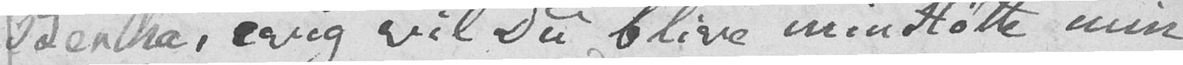Bertha, evig vil Du blive min Støtte min
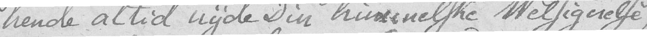hende altid nyde Din himmelske Velsignelse,
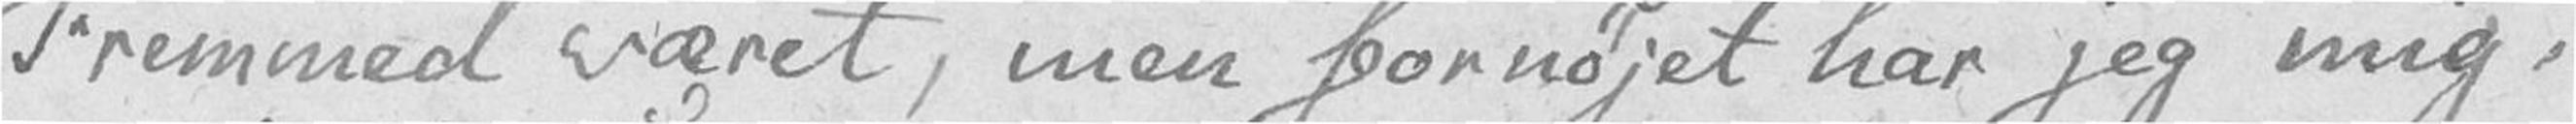Fremmed været, men fornøjet har jeg mig,
